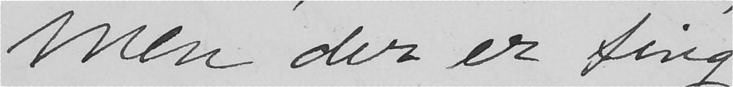men der er ting
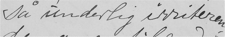så underlig irriteren-
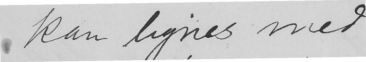kan lignes med
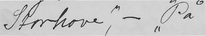Storhove", - "På
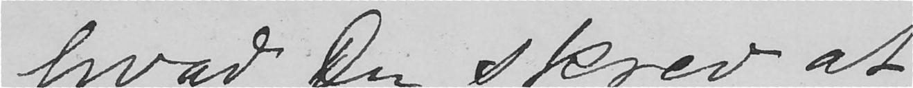hvad Du skrev at
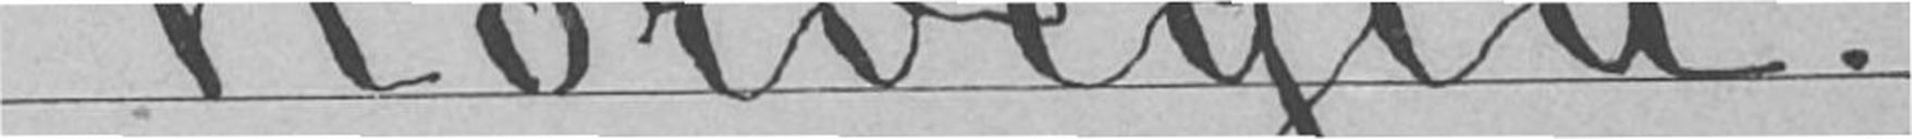Norvegia.
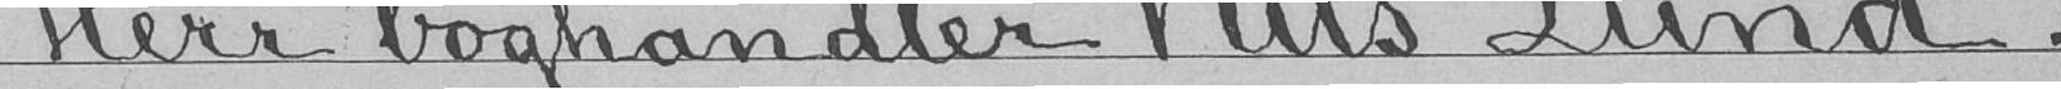Herr boghandler Nils Lund.
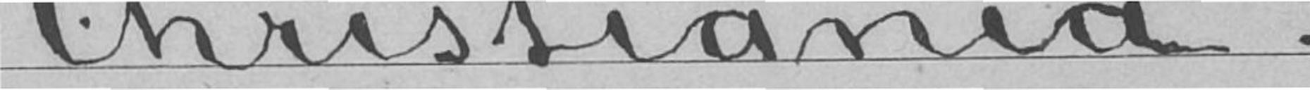Christiania.
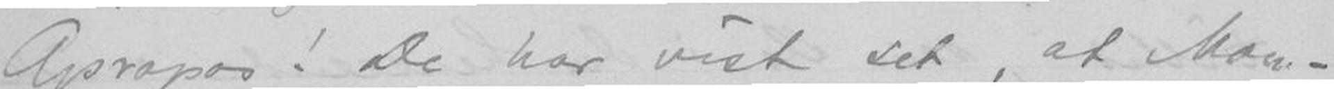Apropos! De har vist set, at Mon-
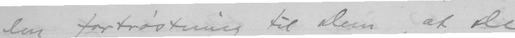den fortrøsning til Dem, at De
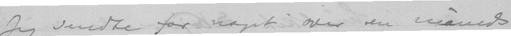Jeg sendte for noget over en måneds
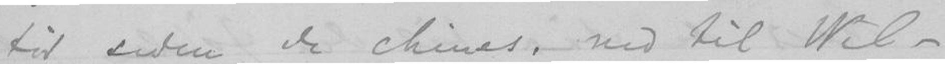tid siden de chines. ned til Wil-
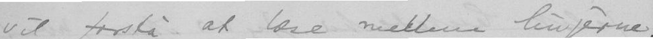vil forstå at læse mellem linjerne,
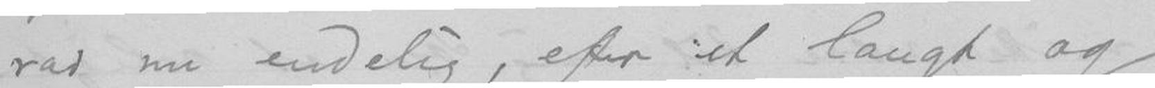rad nu endelig, efter et langt og
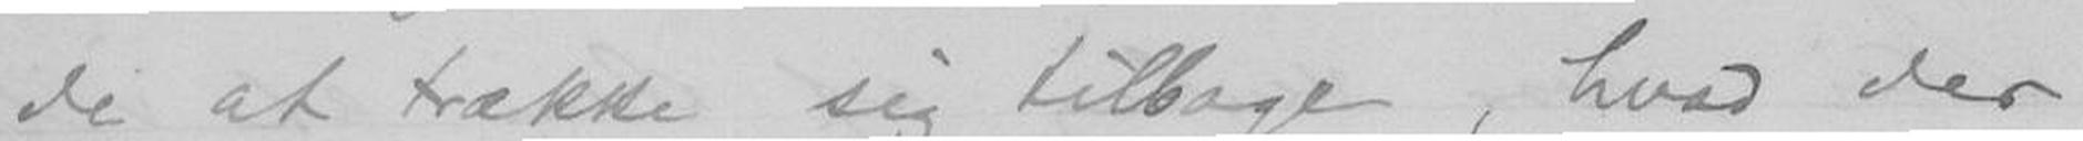de at trække sig tilbage, hvad der
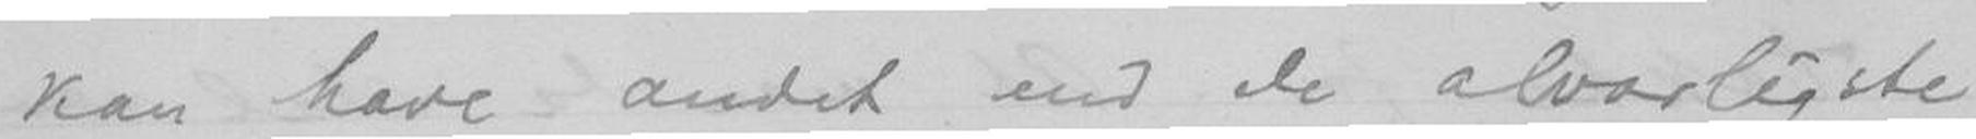kan have andet end de alvorligste
